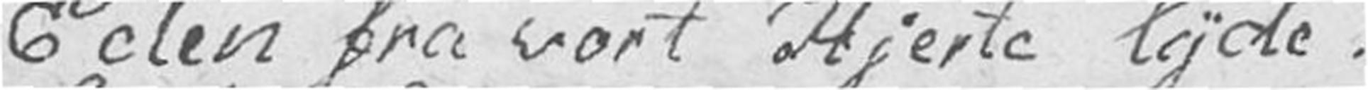Eden fra vort Hjerte lyde!
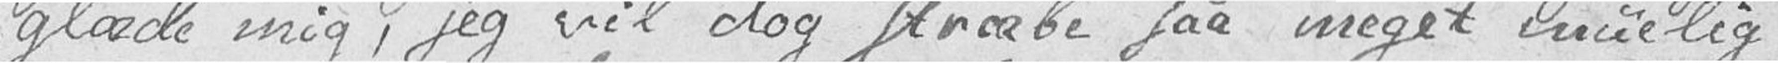glæde mig, jeg vil dog stræbe saa meget muelig
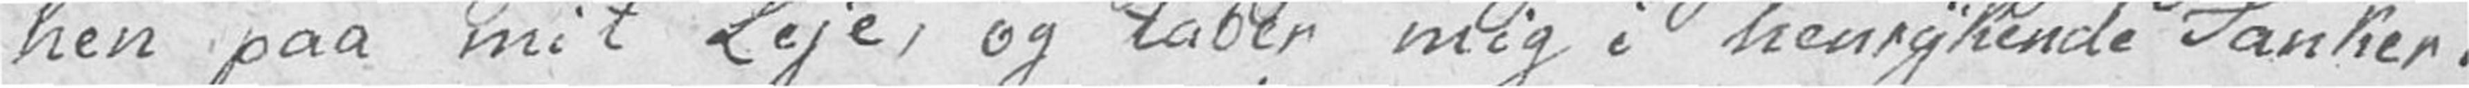hen paa mit Leje, og taber mig i henrykende Tanker.
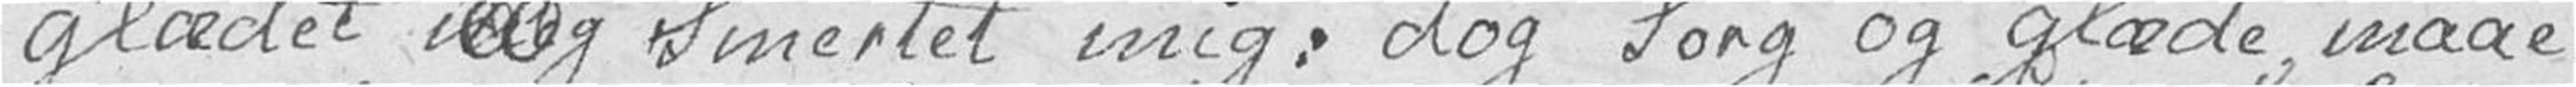glædet og Smertet mig: dog Sorg og glæde maae
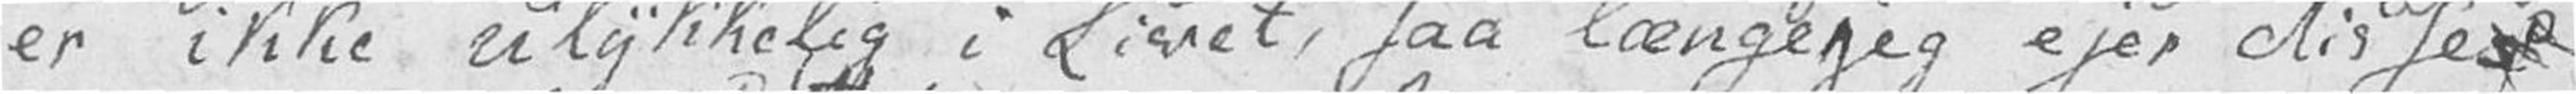er ikke ulykkelig i Livet, saa længe jeg ejer disses
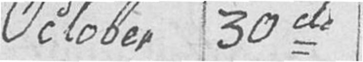October 30de
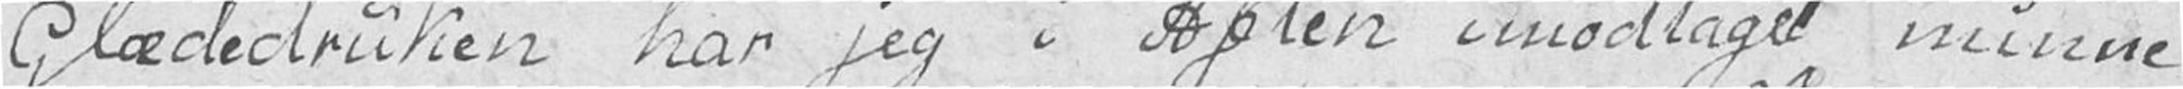Glædedruken har jeg i Aften imodtaget minne
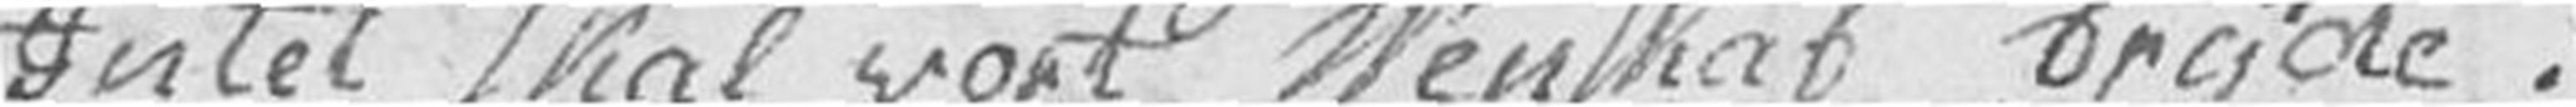Intet skal vort Venskab bryde!
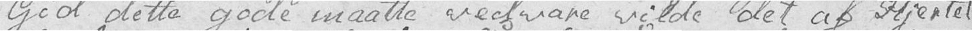Gid dette gode maatte vedvare vilde det af Hjertet
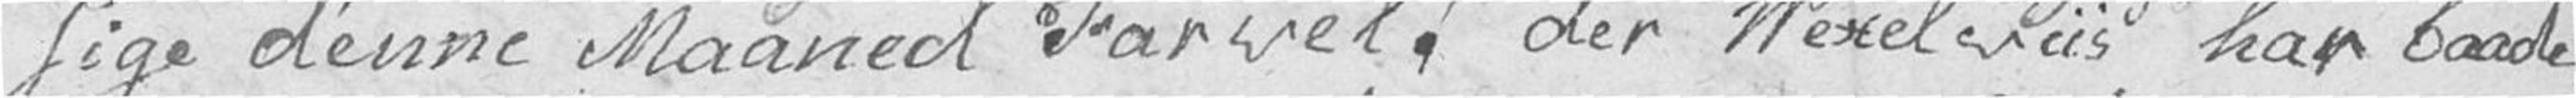sige denne Maaned Farvel! der Vexelviis har baade
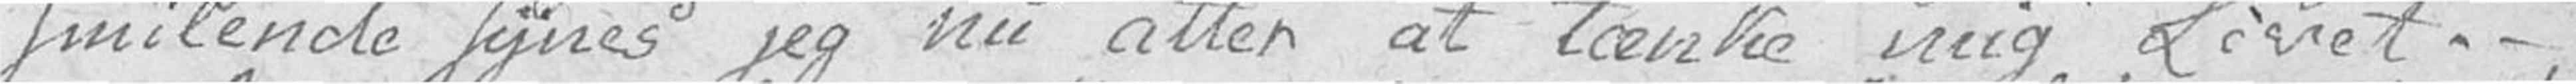smilende synes jeg nu atter at tænke mig Livet. –
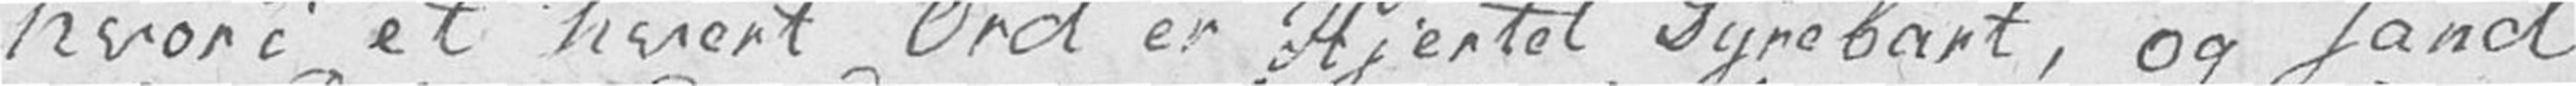hvor i et hvert Ord er Hjertet Dyrebart, og sand
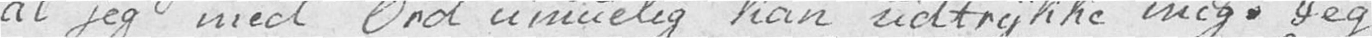at jeg med Ord umuelig kan udtrykke mig. Jeg
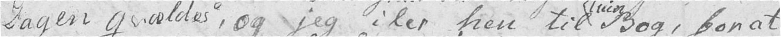Dagen qvældes, og jeg iler hen til min Bog, for at
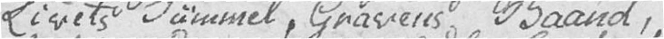Livets Tummel, Gravens Baand,
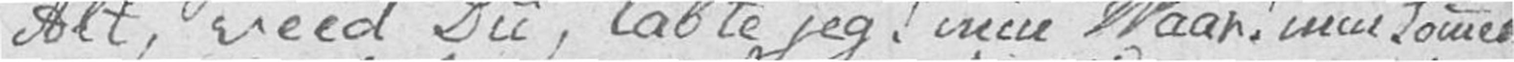Alt, veed Du, tabte jeg! min Vaar! min Sommer
